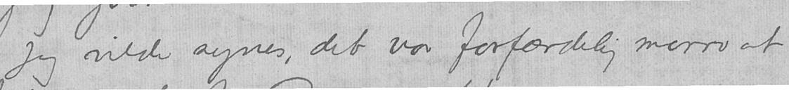Jeg vilde synes, det var forfærdelig morro at
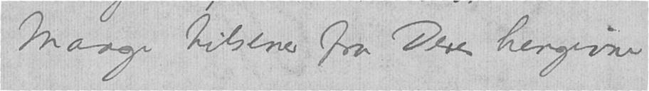Mange hilsener fra Deres hengivne
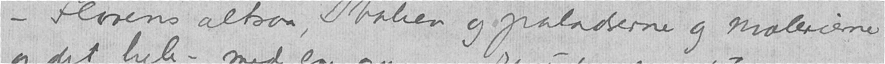– Florens altsaa, Italien og paladserne og malerierne
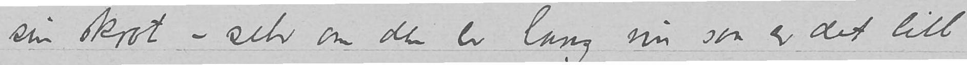sin skrot - selv om den er lang nu saa er det litt
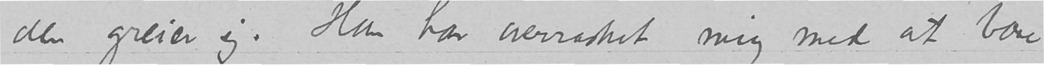den greier sig. Han har overrasket mig med at bære
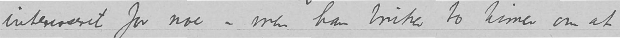interesseret for noe - men han bruker to timer om at
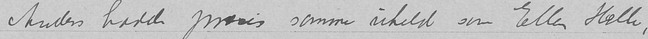Anders hadde præsis samme uheld som Ellen Helle,
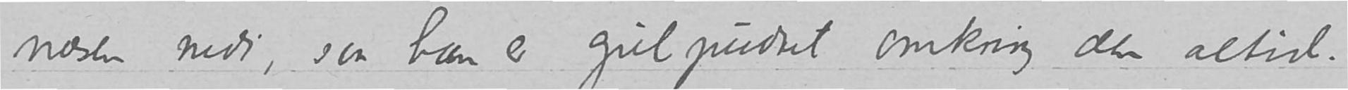næsen nedi, saa han er gulpudret omkring den altid.
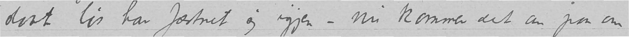slaat løs har fæstnet sig igjen - nu kommer det an paa om
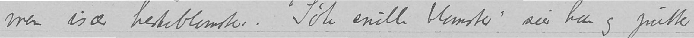men isaær hesteblomster.  «Søte snille blomster» sier han og putter
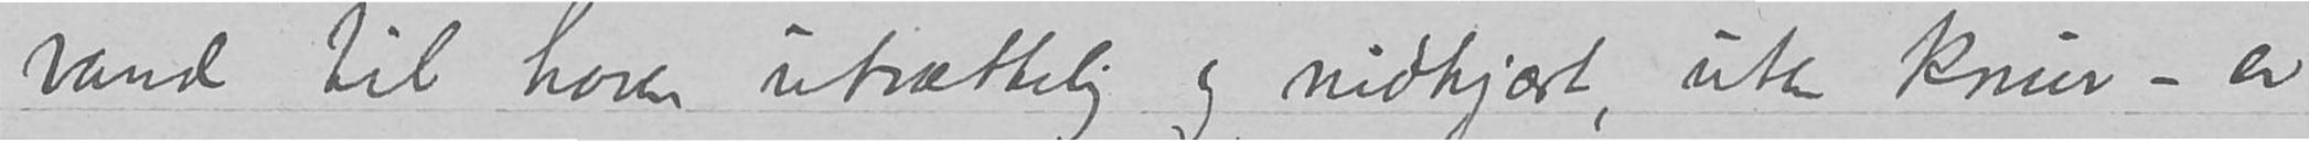vand til haven utrættelig og nidkjært, uten knur - er
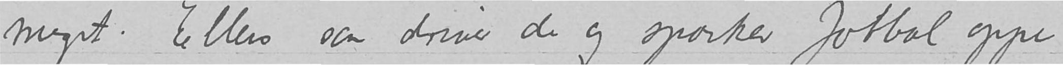meget. Ellers saa driver de og sparker fotbal oppe
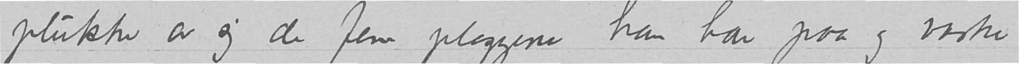plukke av sig de fem plaggene han har paa og vaske
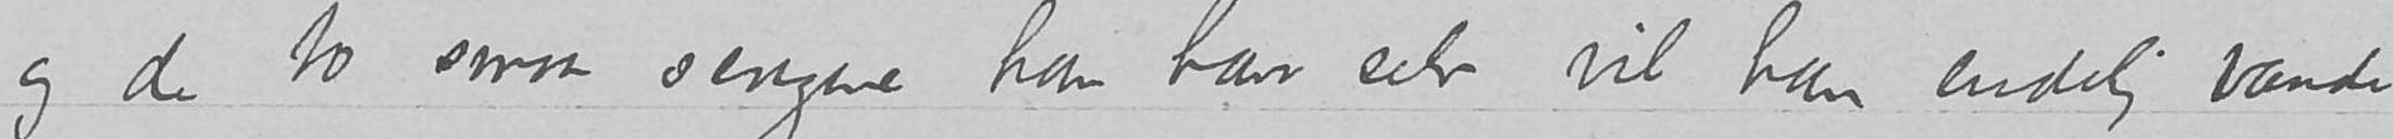og de to smaa sengene han har selv vil han endelig vande
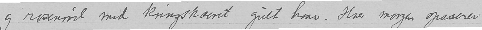og rosenrød med kringskaaret gult haar. Hver morgen spaserer
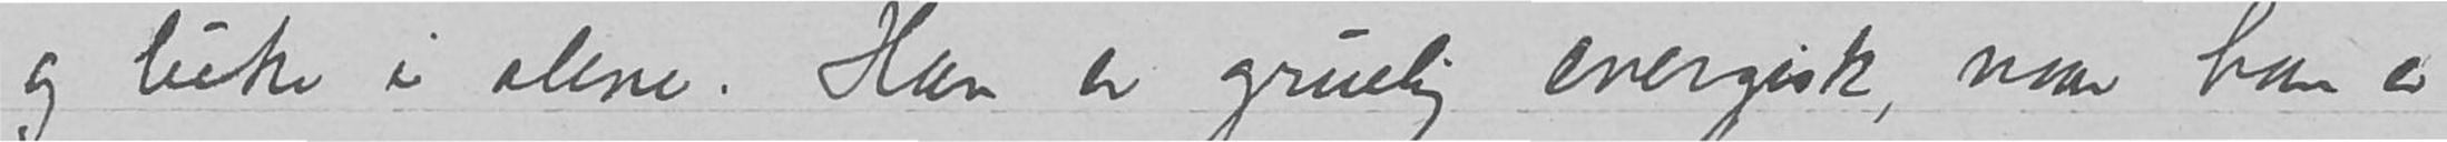og luke i alene.  Han er gruelig energisk, naar han er
